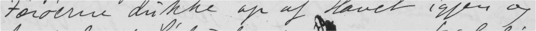Færøerne dukke op af Havet igjen og
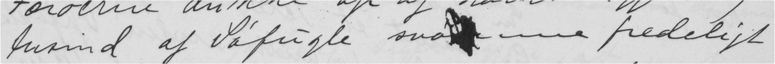tusind af Søfugle svømme fredeligt
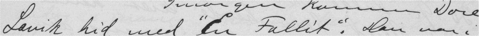Lavik hid med "En Fallit". Han var
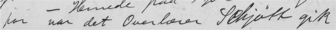for var det Overlærer Schjøtt gik
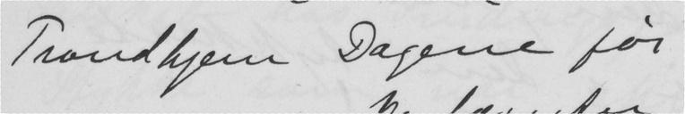Trondhjem Dagene før
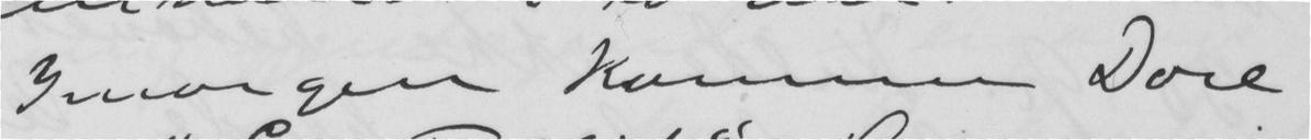Imorgen kommer Dore
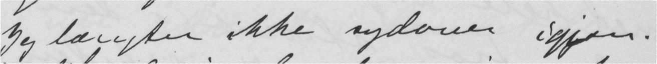Jeg længter ikke sydover igjen.
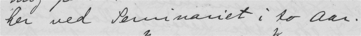her ved Seminariet i to Aar.
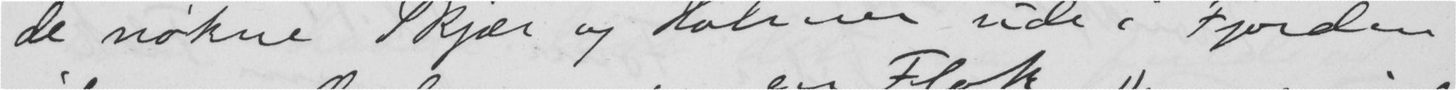de nøkne Skjær og Holmer ude i Fjorden
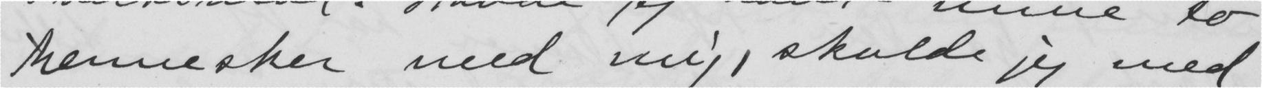Mennesker med mig, skulde jeg med
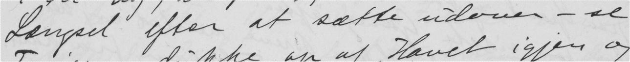Længsel efter at sætte udover - se
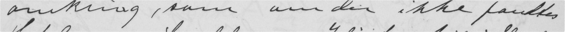omkring, som om der ikke fandtes
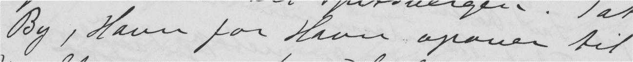By, Havn for Havn opover til
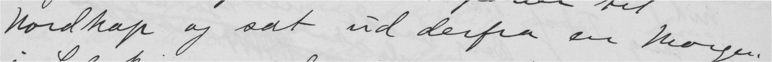Nordkap og sat ud derfra en Morgen
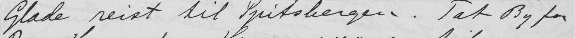Glæde reist til Spitsbergen. Tat By for
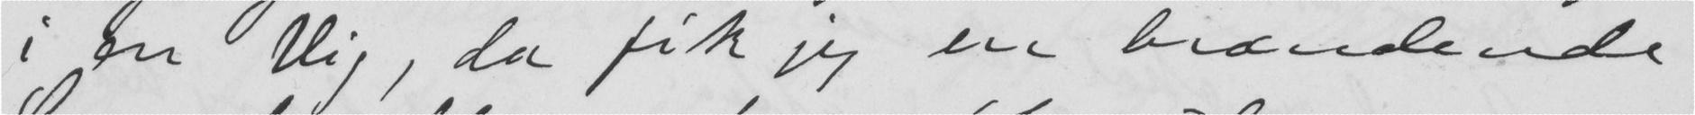i En Vig, da fik jeg en brændende
